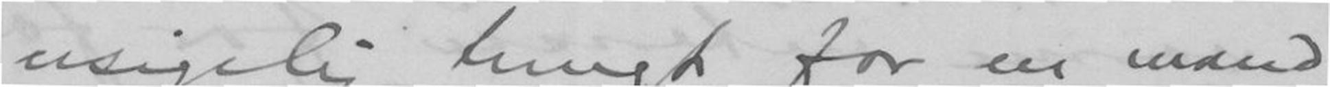usigelig tungt for en mand
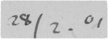28/2.01
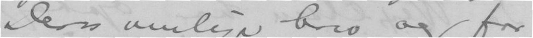Deres venlige brev og for
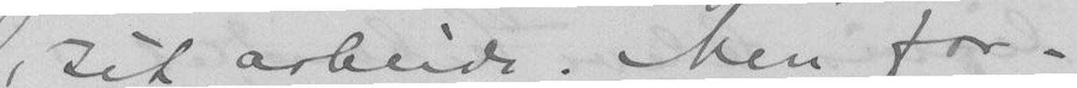i sit arbeid. Men for-
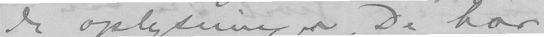de oplysninger, De har
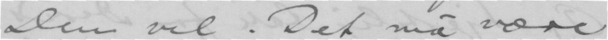Dem vel. Det må være
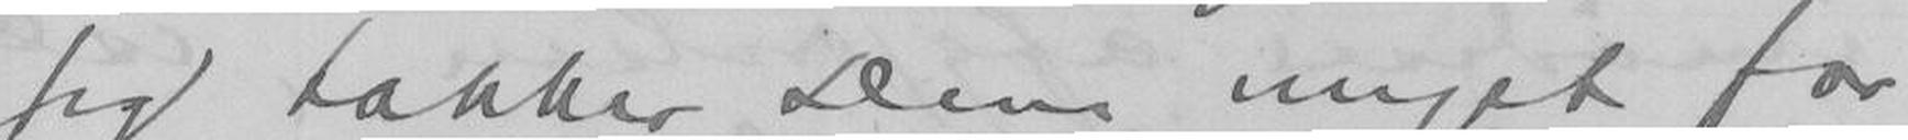Jeg takker Dem meget for
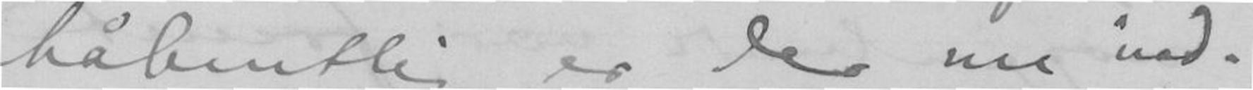håbentlig er der nu ind-
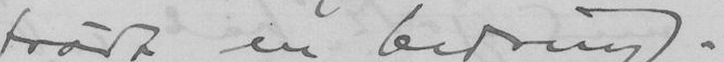trådt en bedring.
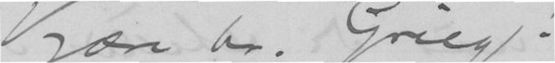Kjære hr. Grieg!
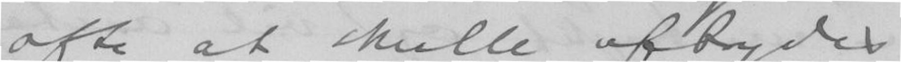ofte at skulle afbrydes
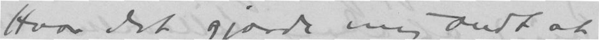Hvor det gjorde mig ondt at
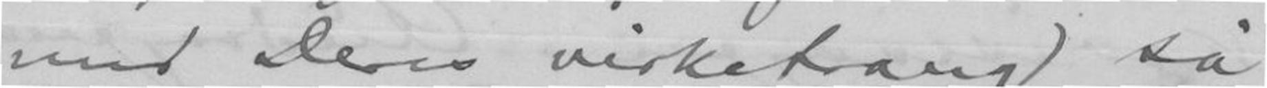med Deres virketrang så
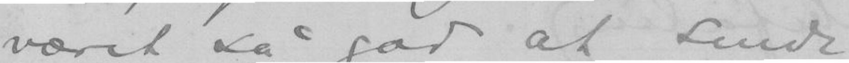været så god at sende
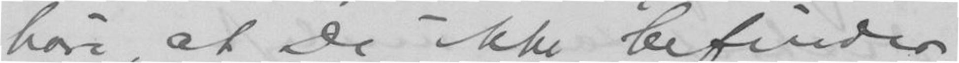høre, at De ikke befinder
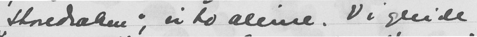Storedraken; vi to alene. Vi oplevde
